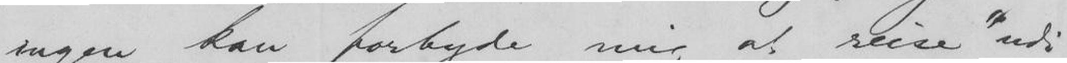ingen kan forbyde mig at reise "udi
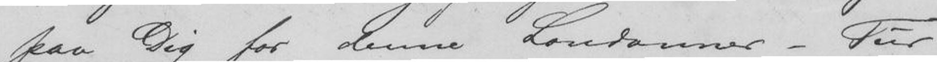paa Dig for denne Londonner-Tur,
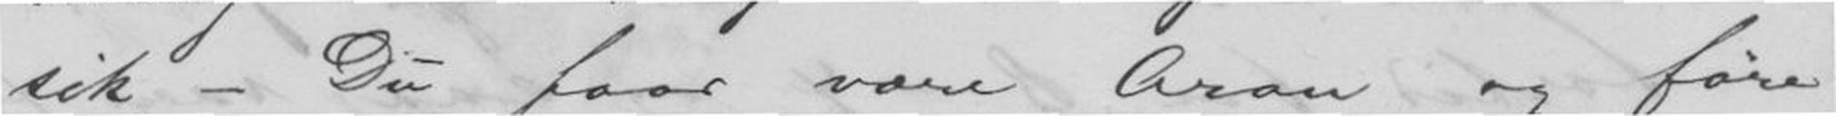sik - Du faar være Aran og føre
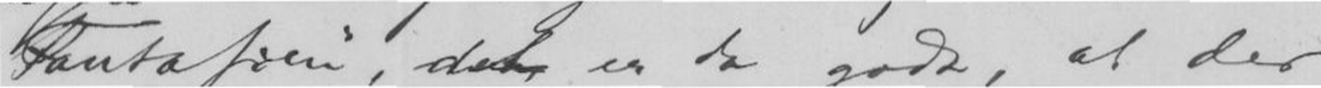Fantasien", det er da godt, at der
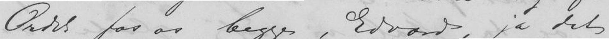Ordet for os begge, Edvard, ja det
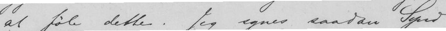at føle dette. Jeg synes saadan Synd
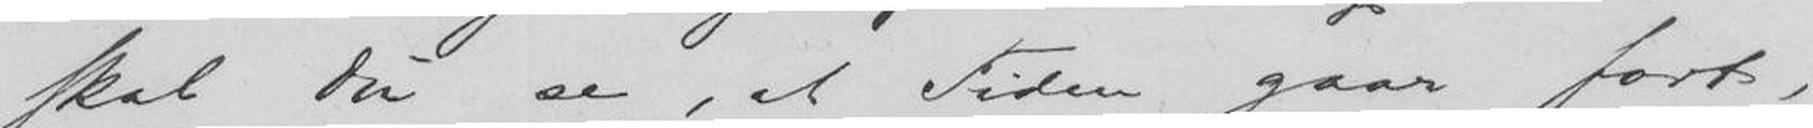skal du se, at Tiden gaar fort,
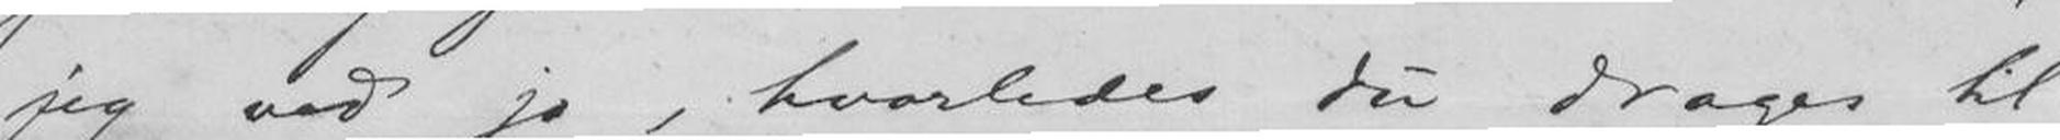jeg ved jo, hvorledes Du drages til
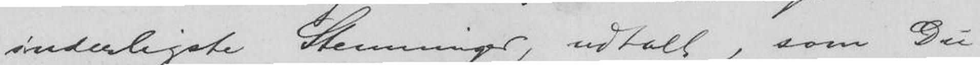inderligste Stemninger, udtalt, som Du
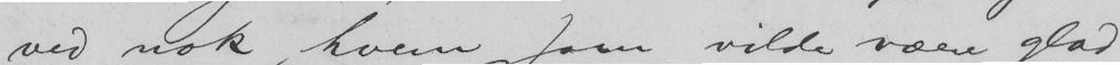ved nok, hvem som vilde være glad
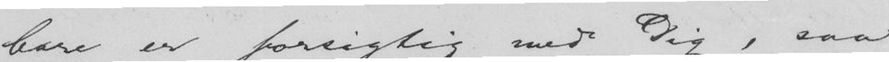bare er forsigtig med Dig, saa
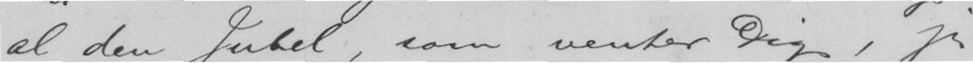al den Jubel, som venter Dig, jeg
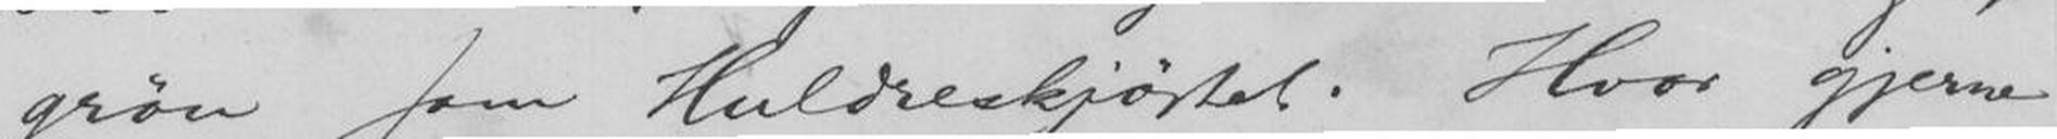grøn som Huldreskjørtet. Hvor gjerne
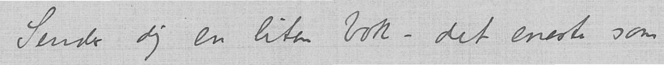Sender dig en liten bok – det eneste som
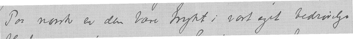Paa norsk er den bare trykt i vort eget bedrøvelige
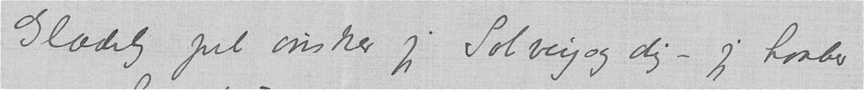Glædelig jul ønsker jeg Solveig og dig – jeg haaber
